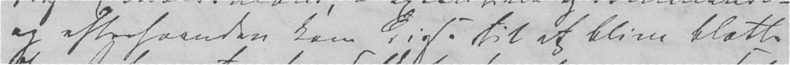og efterhaanden kom disse til at blive blotte
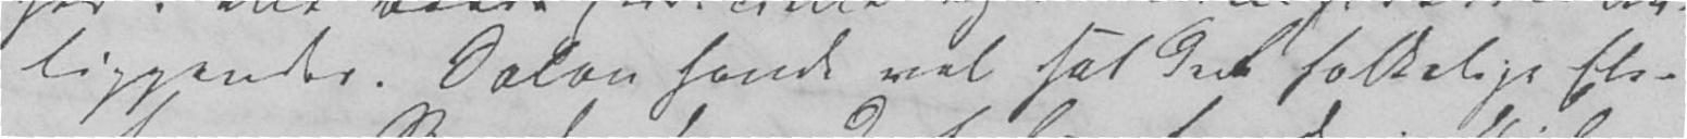liggender. Solon havde vel sat det folkelige Ele-
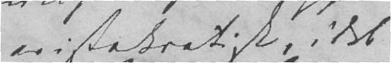aristokratisk, idet
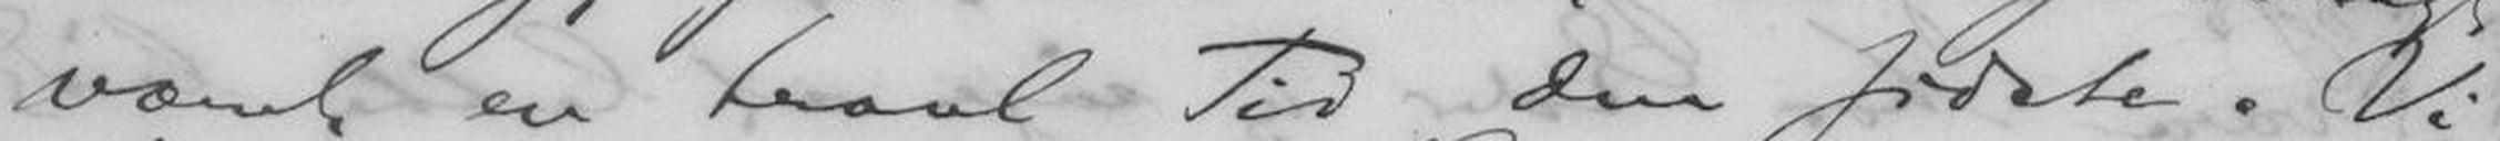været en travel Tid den sidste. Vi
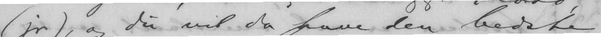(jr), og da vil da have den bedste
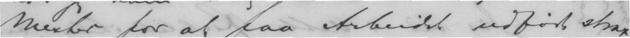Mester for at faa Arbeidet udført strax
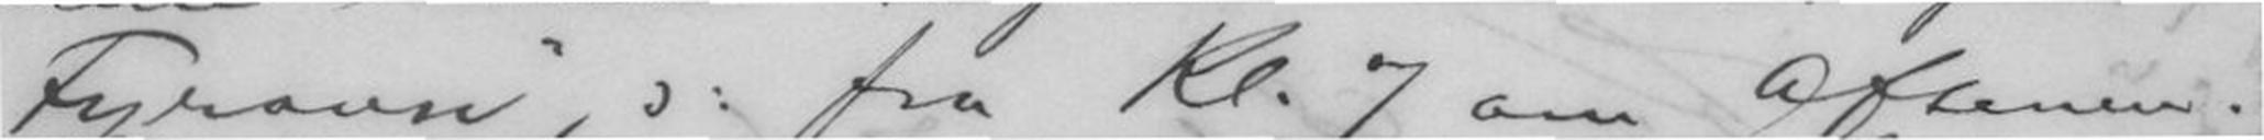Fyravn", ): fra Kl. 7 om Aftenen.
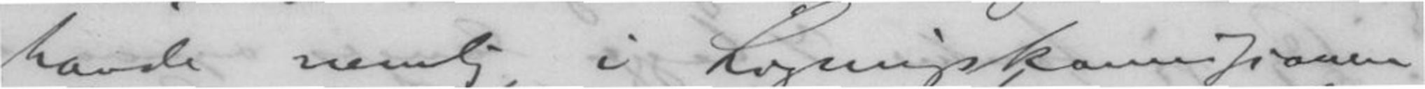havde nemlig i Ligningkommissionen
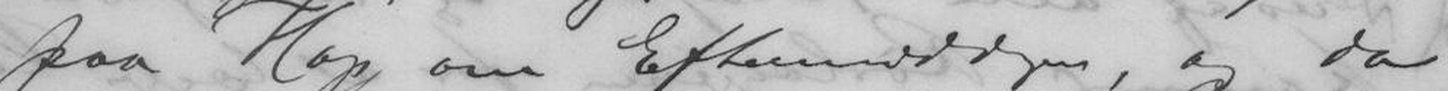paa Hop om Eftermiddagen, og da
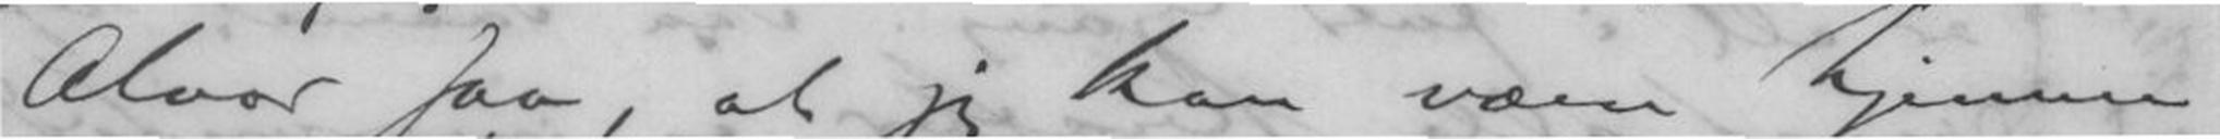Alvor saa, at jeg kan være hjemme
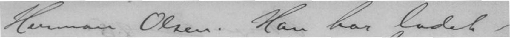Herman Olsen. Han har ladet
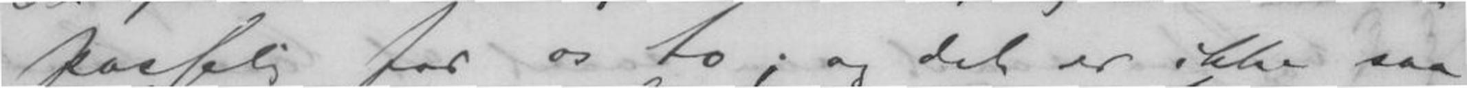passelig for os to; og det er ikke saa
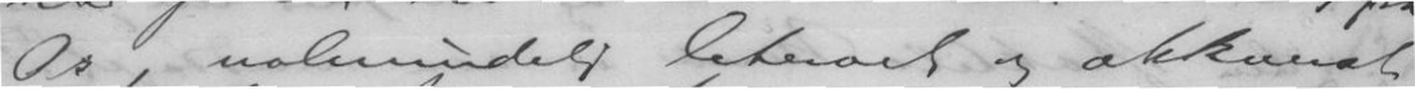Os, ualmindelig letroet og akkurat
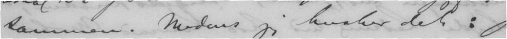sammen. Medens jeg husker det: jeg
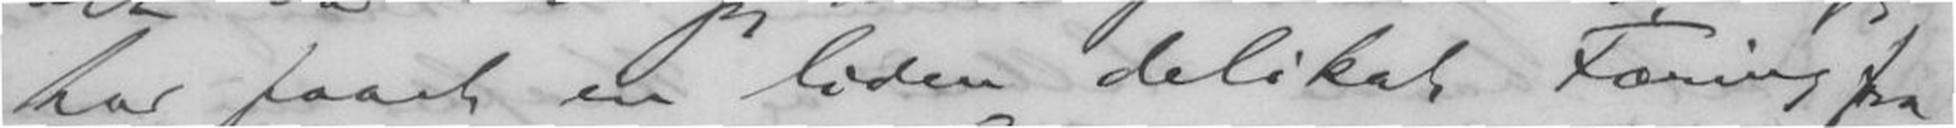har faaet en liden delikat Færing fra
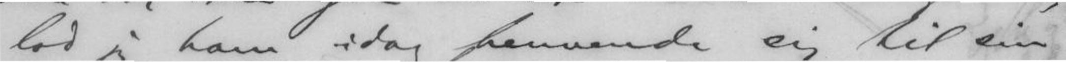lod jeg ham idag henvende sig til sin
